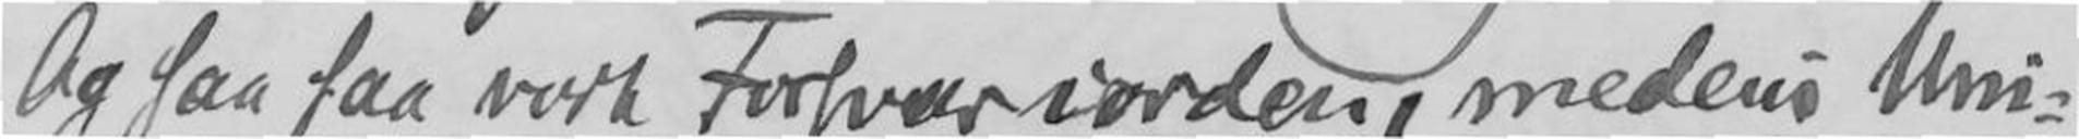Og saa faa vort Forsvar iorden, medens Uni-
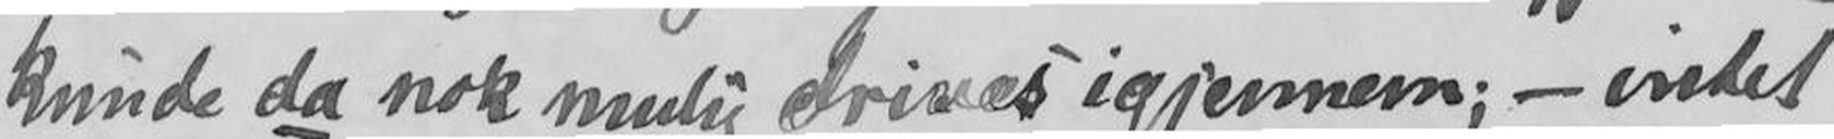kunde da nok mulig drives igjennem; - intet
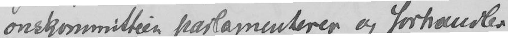onskommitteen parlamenterer og forhandler
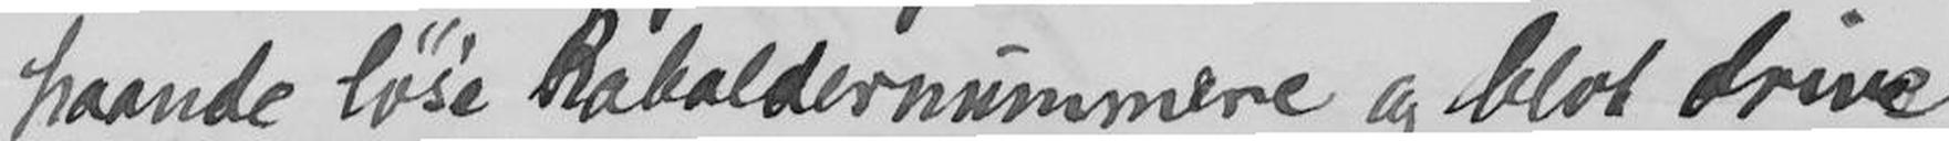haande løse Rabaldernummere og blot drive
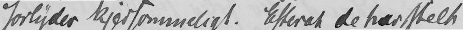forlyder kjedsommeligt. Efterat de har stelt
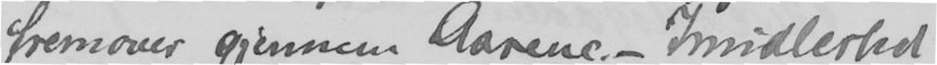fremover gjennem Aarene. - Imidlertid
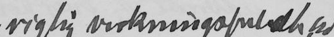rigtig virkningsfuldt ud
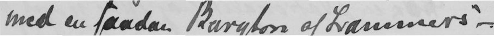med en saadan Baryton af Lammers -
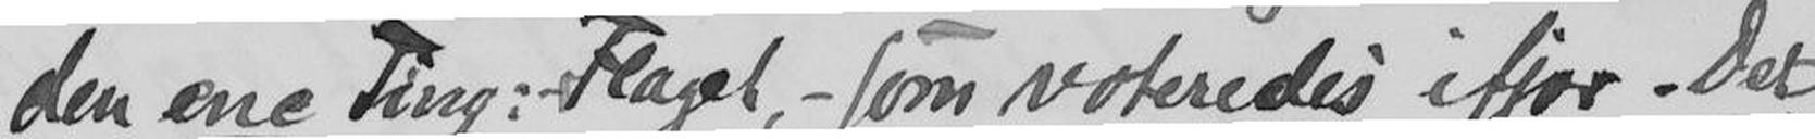den ene Ting: Flaget, - som voteredes ifjor. Det
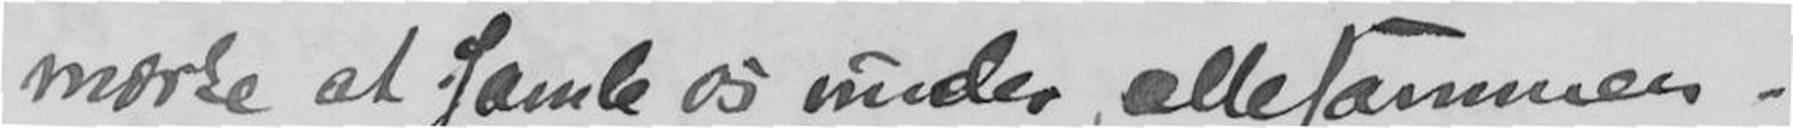mærke at samle os under allesammen.
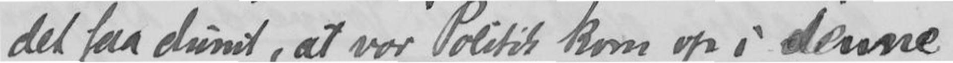det saa dumt, at vor Politik kom op i denne
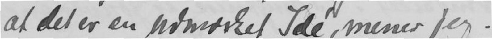at det er en udmærket Ide, mener jeg.
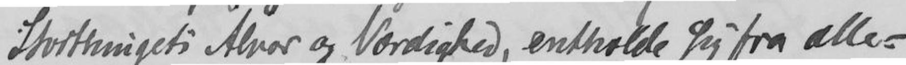Storthingets Alvor og Værdighed, entholde sig fra alle-
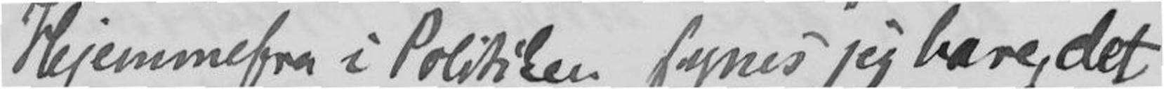Hjemmefra i Politiken synes jeg bare det
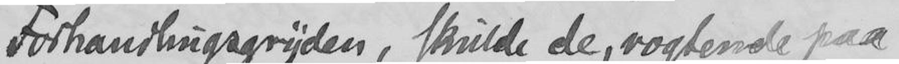Forhandlingsgryden, skulde de, vogtende paa
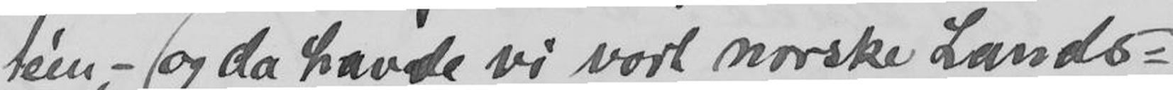teen, - og da havde vi vort norske Lands-
